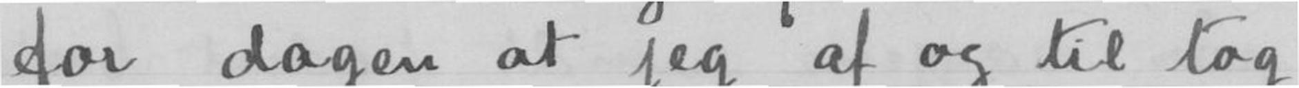for dagen at jeg af og til tog
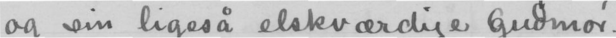og sin ligeså elskværdige Gudmo'r.
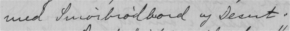med Smørbrødbord og Desert.
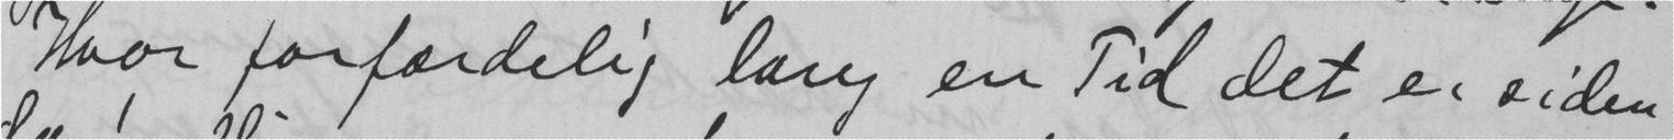Hvor forfærdelig lang en Tid det er siden
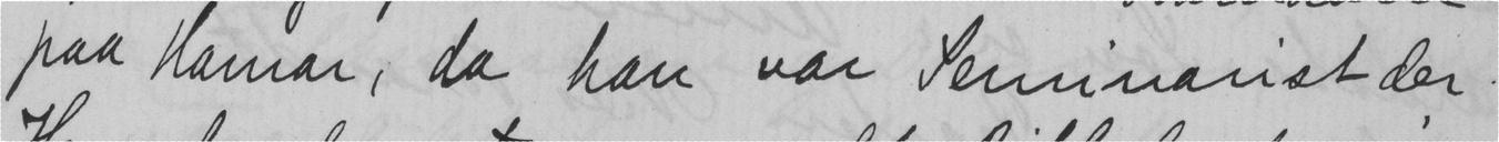paa Hamar, da han var Seminarist der.
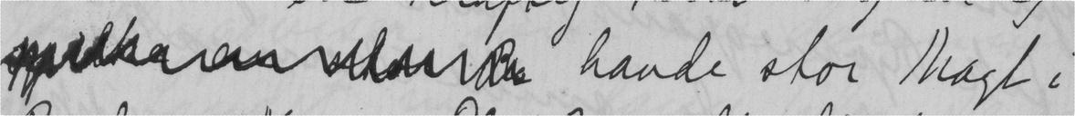havde stor Magt i
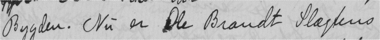Bygden. Nu er Ole Brandt Slægtens
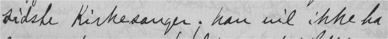sidste Kirkesanger; han vil ikke ha
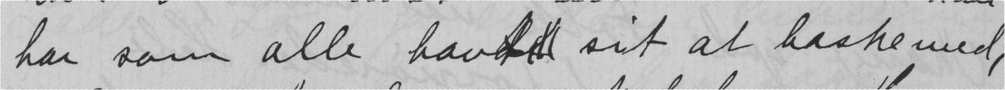har som alle havt  sit at haske med,
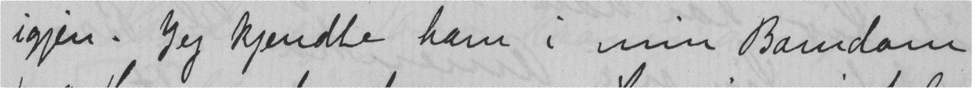igjen. Jeg kjendte ham i min Barndom
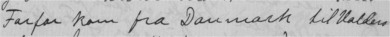Farfor kom fra Danmark til Valders
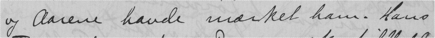og Aarene havde mærket ham. Hans
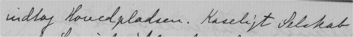indtog Hovedpladsen. Koseligt Selskab
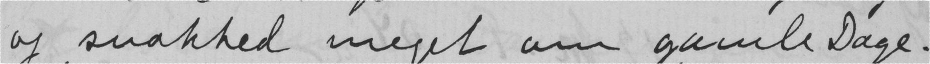og snakked meget om gamle Dage.
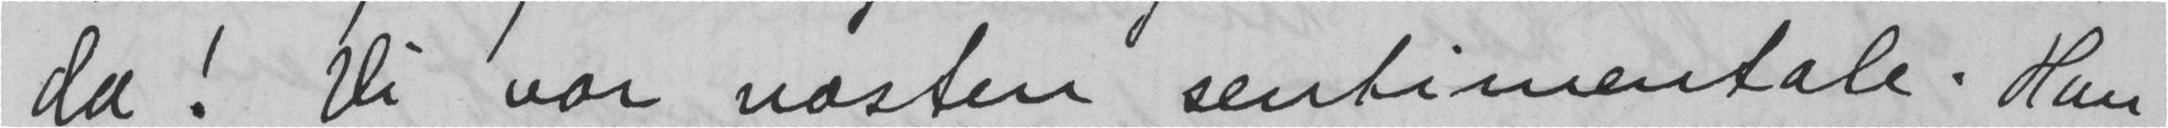da! Vi var næsten sentimentale. Han
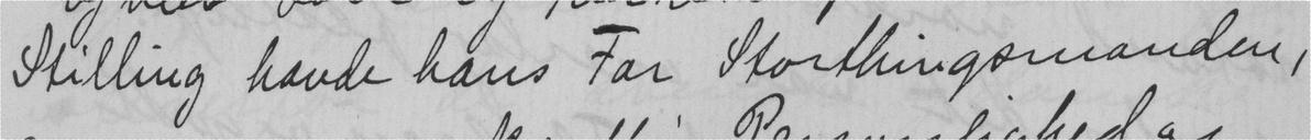Stilling havde hans Far Storthingsmanden,
